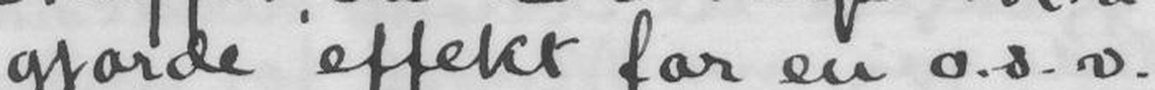gjorde effekt for en o.s.v.
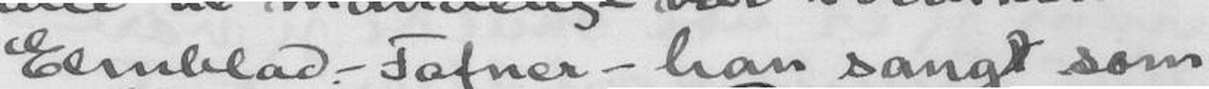Eernblad, - Fafner - han sang som
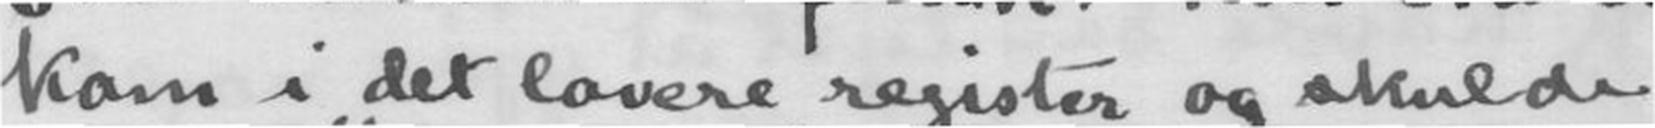kom i det lavere register og skulde
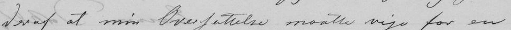deraf at min Oversættelse maatte vige for en
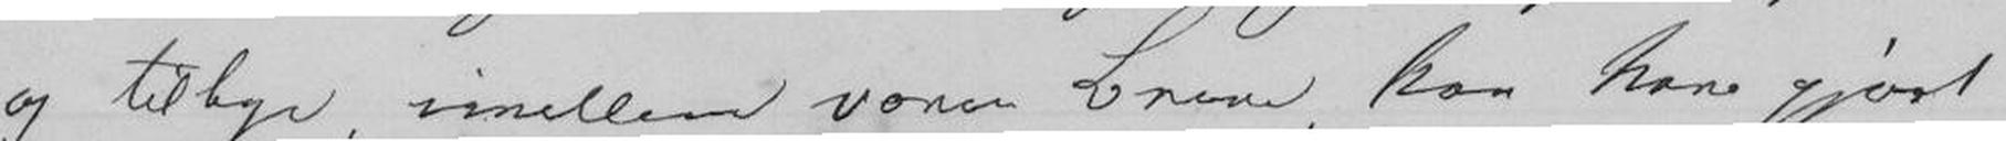og tilyr, imellem vore Liene, kan have gjort
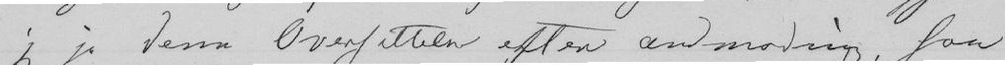jeg jo denne Oversettelsen efter andmodning, saa
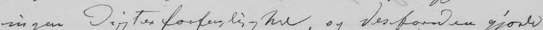ingen Digterforferlighed, og desforuden gjorde
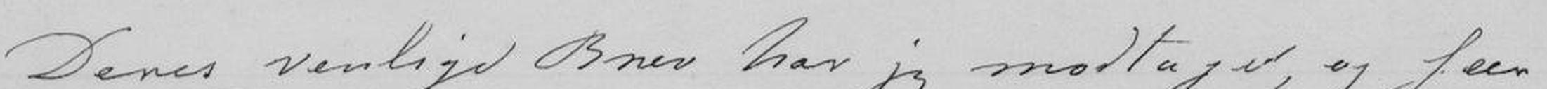Deres venlige Brev har jeg modtaget, og seer
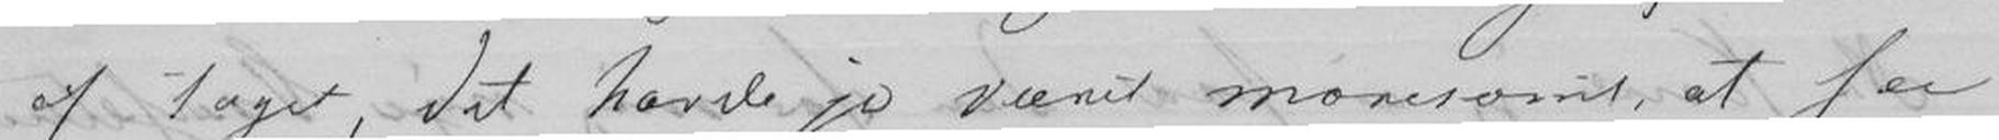af taget, det havde jo været moresomt, at se
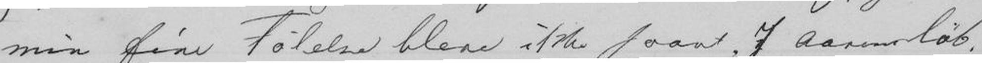min fine Følelse bleve ikke saart. I aarenesløb
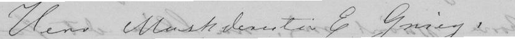Herr Muskdernter E Grieg,
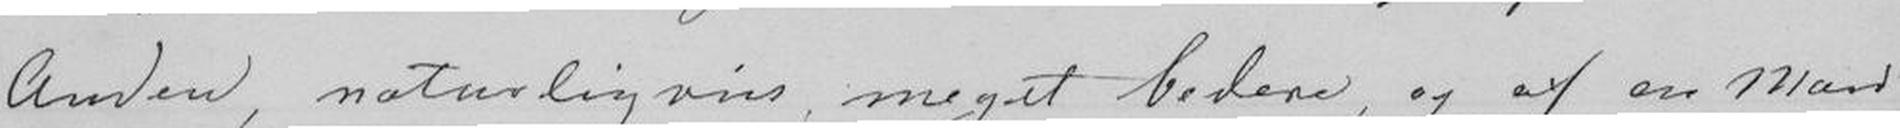Anden, naturligvis meget bedere, og af en Mand
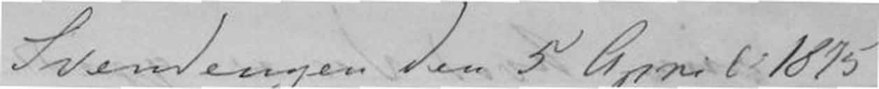Svendengen den 5 April 1875
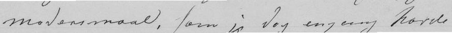modersmaal, som jeg dog engang havde
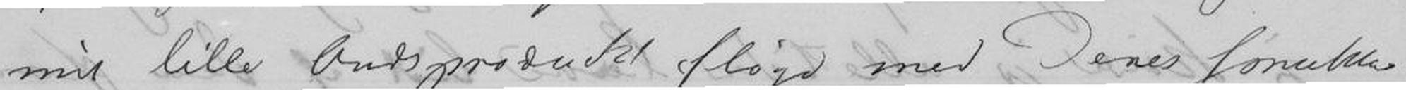mit lilla Åndsprodukt fløgd med Deres smukke
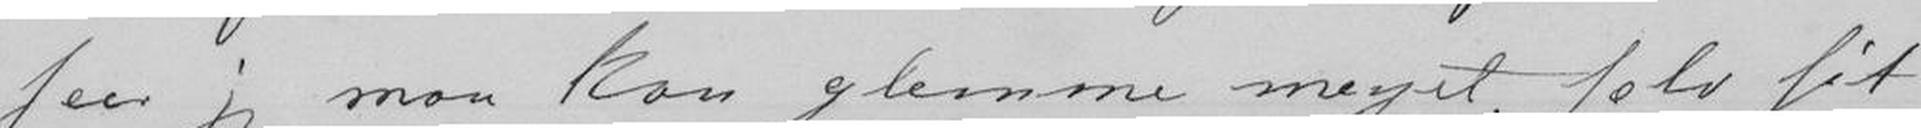ser jeg man kan glemme meget, selv sit
